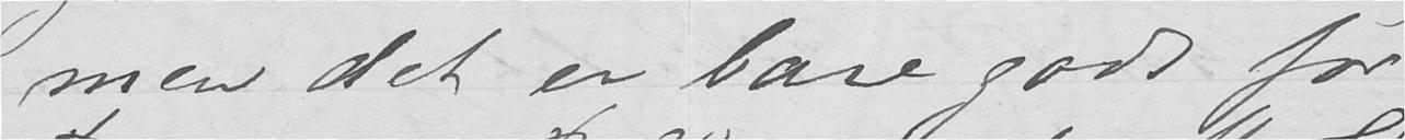men det er bare godt for
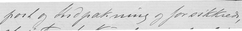port og Indpakning og forsikkrede,
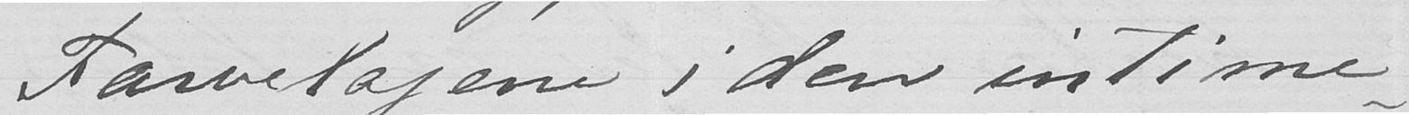Farvelagene i den intime-
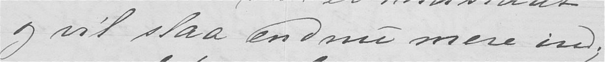og vil slaa endnu mere ind;
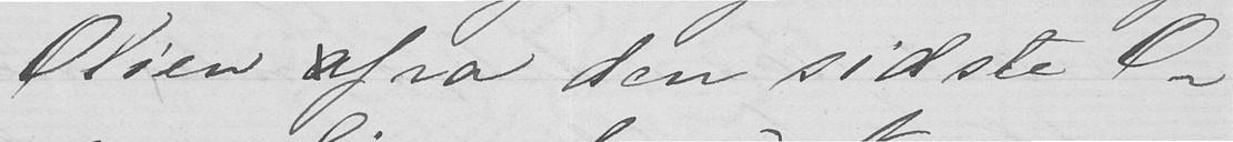Olien afra den sidste O-
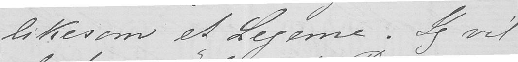likesom et Legeme. Jeg vil
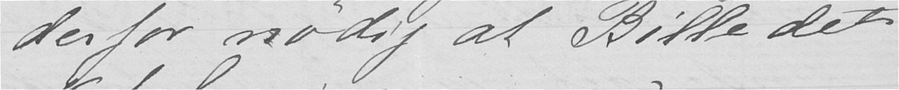derfor nødig at Billedet
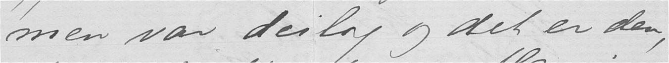men var deilig og det er den,
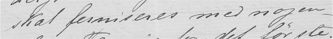skal ferniseres med nogen-
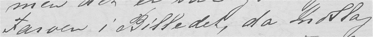Farven i Billedet, da Indslag
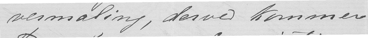vermaling, derved kommer
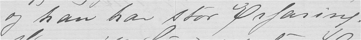og han har stor Erfaring.
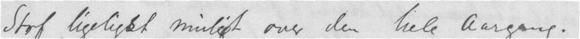Stof ligeligst muligt over den hele Aargang.
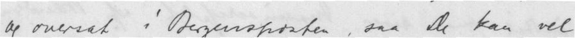og oversat i Bergensposten saa De kan vel
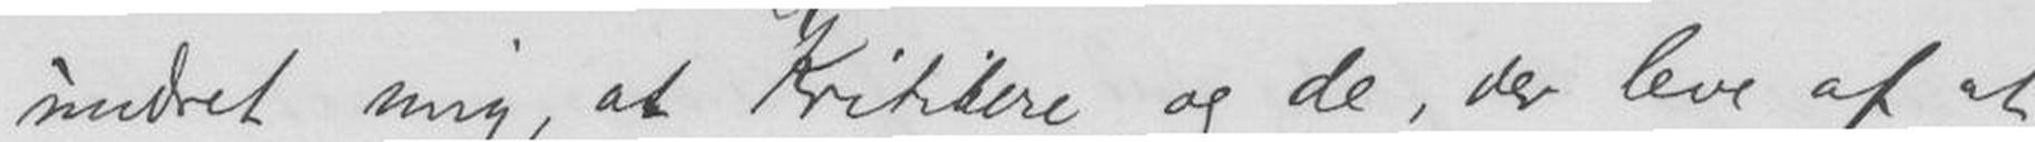undret mig, at Kritikere if de, de lever af at
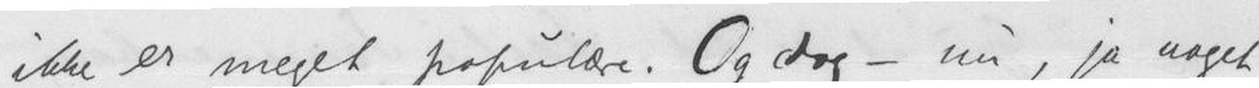ikke er meget populære. Og dog - vi, ja noget
